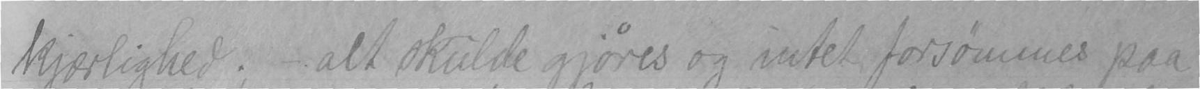kjærlighed; - alt skulde gjøres og intet forsømmes paa
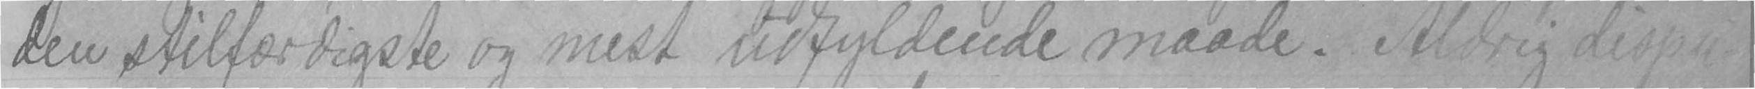den stilfærdigste og mest udfyldende maade. Aldrig dispu-
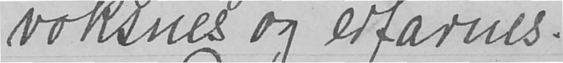voksnes og erfarnes.
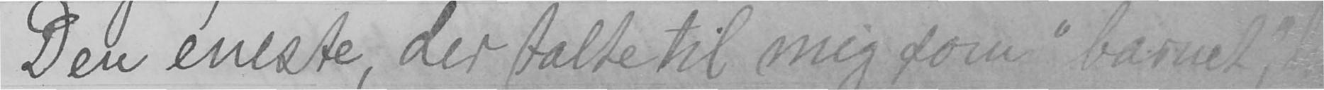Den eneste, der talte til meg som "barnet",
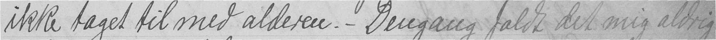ikke taget til med alderen. - Dengang faldt det mig aldrig
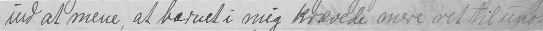ind at mene, at barnet i mig krævede mere vet til uno-
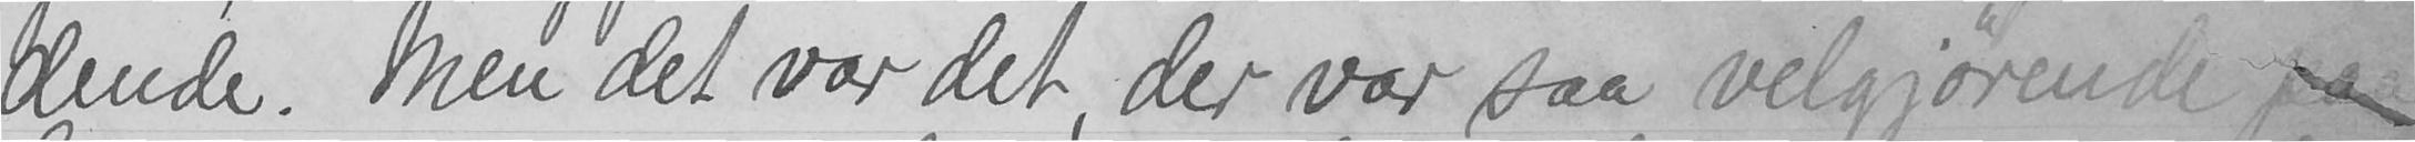dende. Men det var det, der var saa velgjørende paa
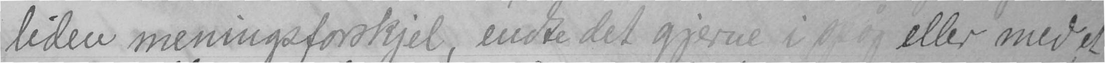liden meningsforskjel, endte det gjerne i spøg eller med et
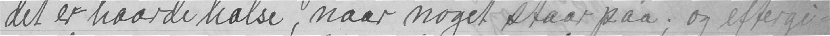det er haarde halse, naar noget staar paa; og eftergi-
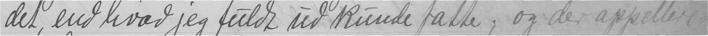det, end hvad jeg fuldt ud kunde fatte; og der appellere-
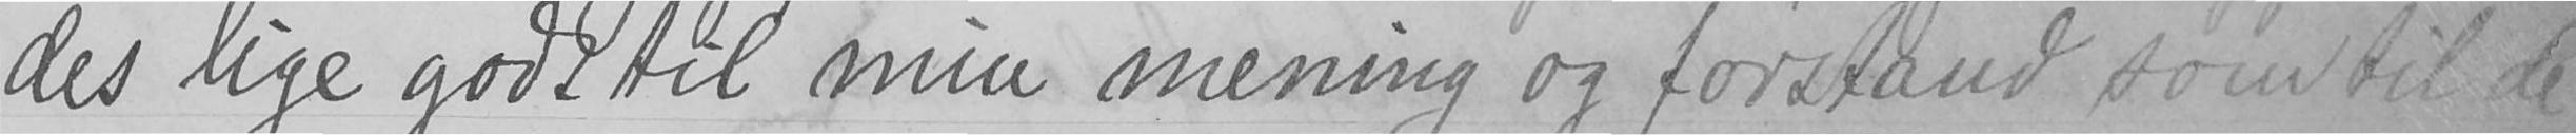des lige godt til min mening og forstand som til de
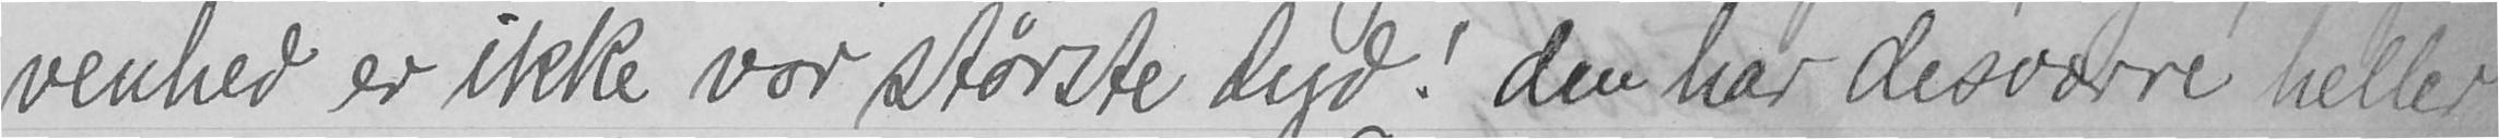venhed er ikke vor største dyd! den har desværre heller
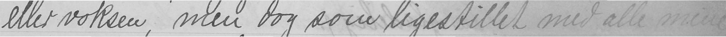eller voksen, men dog som ligestillet med alle mine
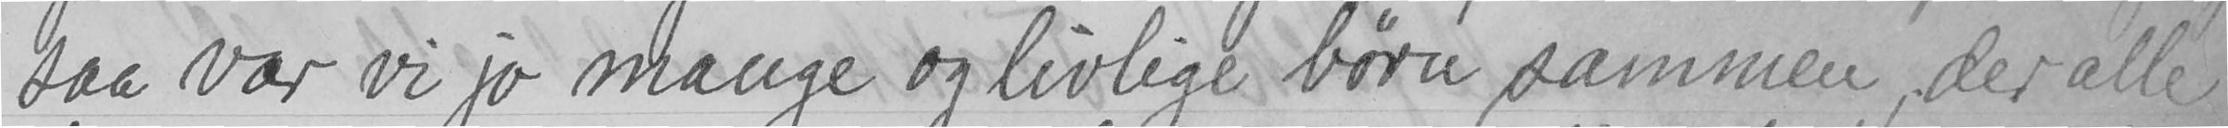saa var vi jo mange og livlige børn sammen, der alle
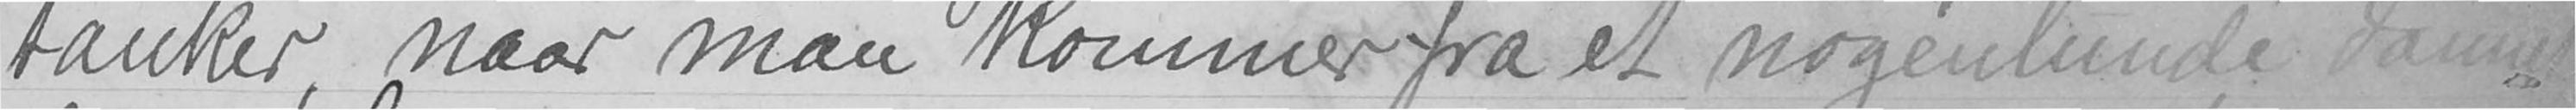tanker, naar man kommer fra et nogenlunde dannet
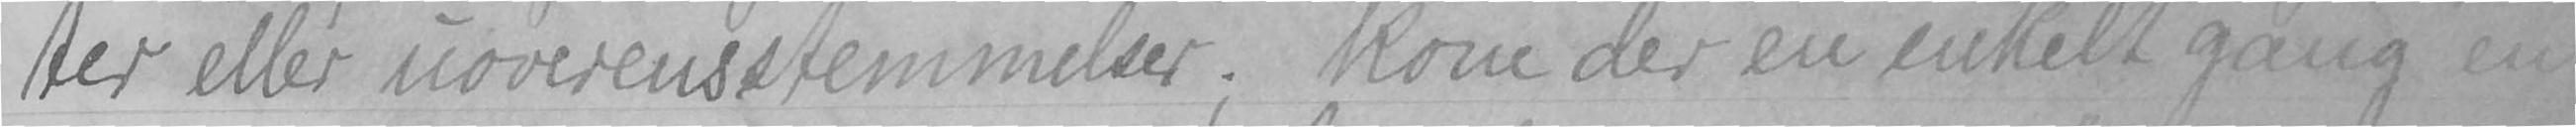ter eller uoverensstemmelser; kom der en enkelt gang en
